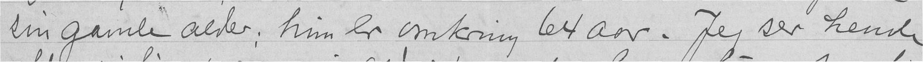sin gamle alder; hun er omkring 64 aar. Jeg ser hende
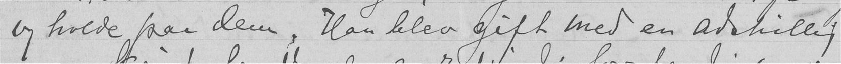og holde paa dem. Han blev gift med en adskillig
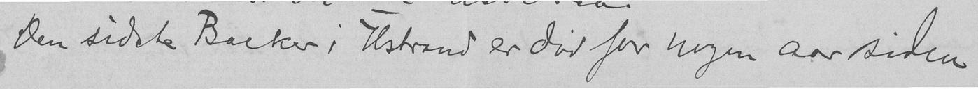Den sidste Backer i Hstrand er død for nogen aar siden.
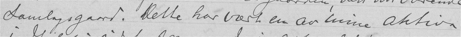Samlagsgaard. Dette har vært en av mine aktiva -
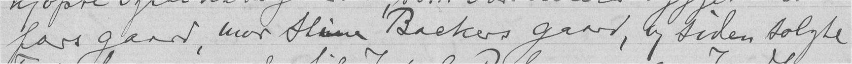fars gaard, hvor Stine Backers gaard, og siden solgte
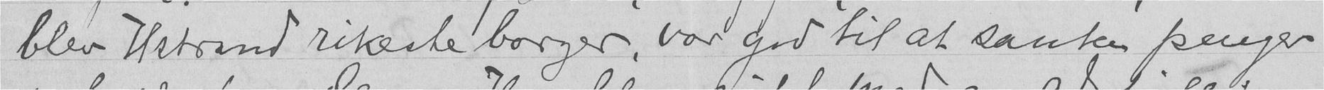blev Hstrand rikeste borger, var god til at sanke penger
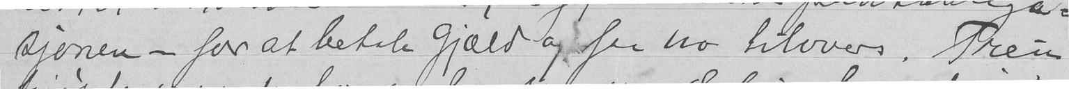sjonen - for at betale gjæld og faa no tilovers. Treu
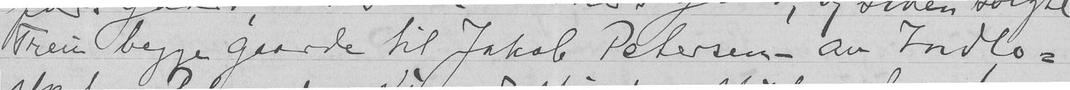Treu begge gaarde til Jakob Petersen - av Indlo-
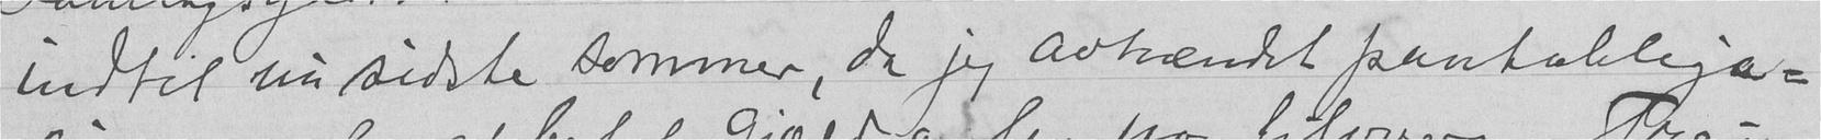indtil nu sidste sommer, da jeg avhændet pantobliga-
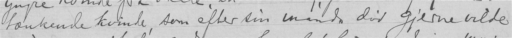tænkende kvinde, som efter sin mands død gjerne vilde
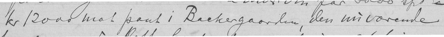kr 12000 mot pant i Backergaarden, den nuværende
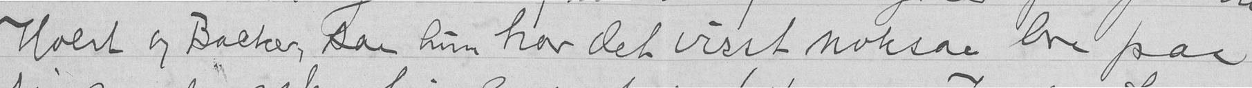Holst og Backer, saa hun har det visst noksaa bra paa
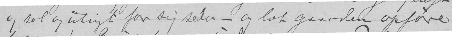og sol og utsigt for sig selv - og lot gaarden opføre
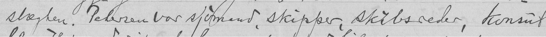slægten. Petersen var sjømand, skipper, skibsreder, konsul,
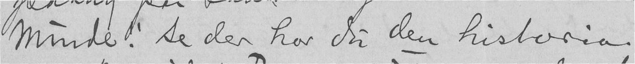Minde." Se der har du den historia.
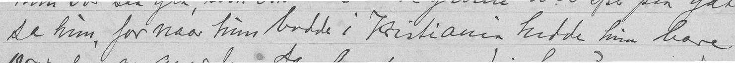sa hun, for naar hun bodde i Kristiania hadde hun bare
